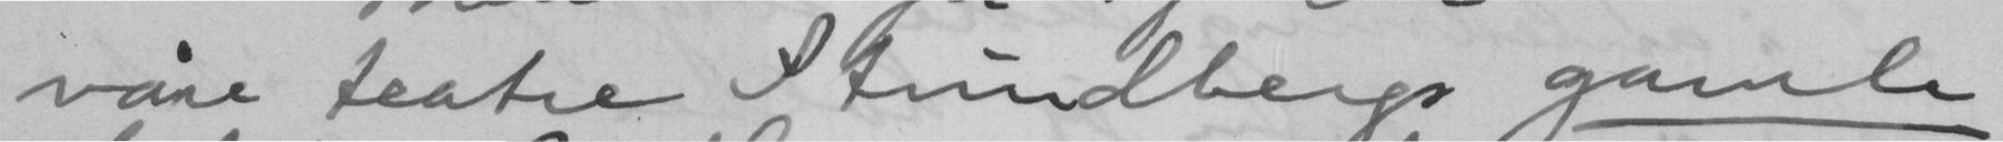våre teatre Strindbergs gamle
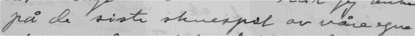på de siste skuespil av våre egne
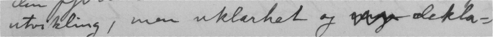utvikling, men uklarhet og  dekla-
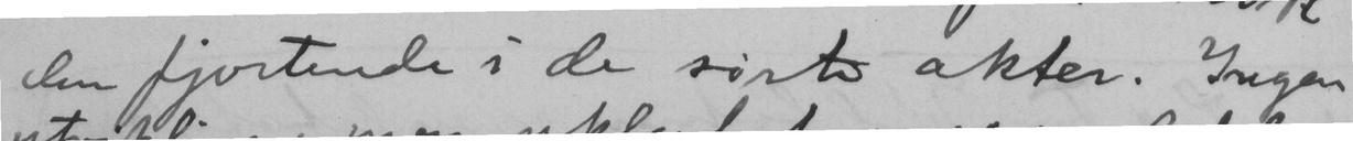den fjortende i de sist akter. Ingen
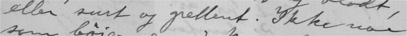eller surt og grellent. Ikke noe
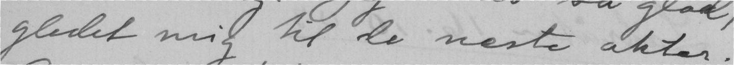gledet mig til de neste akter.
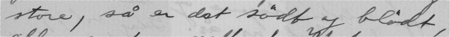store, så er det sødt og blødt,
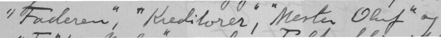"Faderen", "Kreditorer", "Mester Oluf" og
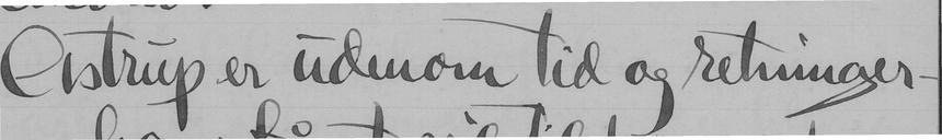Astrup er udenom tid og retninger -
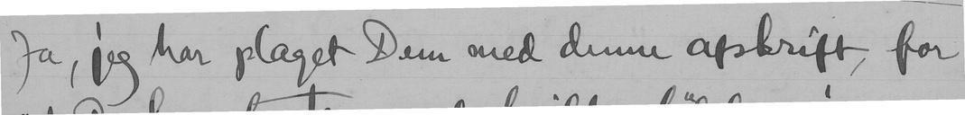Ja, jeg har plaget Dem med denne afskrift, for
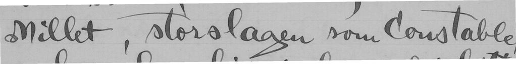Millet, storslagen som Constable,
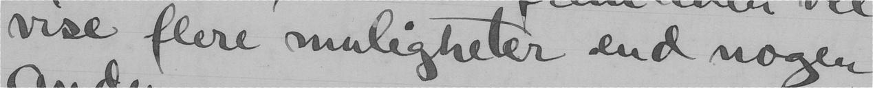vise flere muligheter end nogen
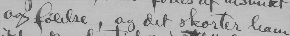og følelse, og det skorter ham
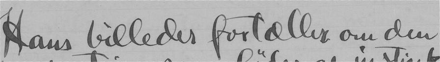Hans billeder fortæller om den
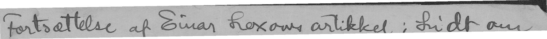Fortsættelse af Einar Lexows artikkel: Lidt om
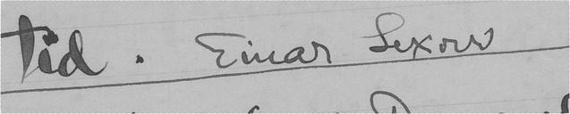tid. Einar Lexow
